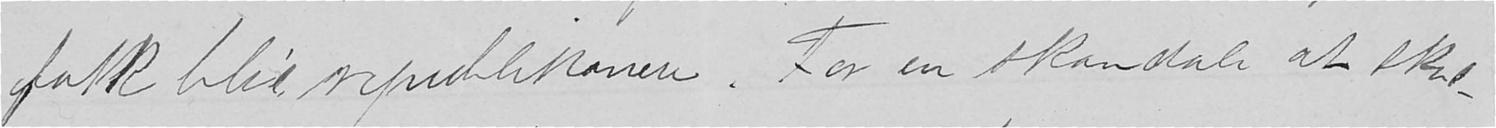folk blive republikanere. For en skandale at skul-
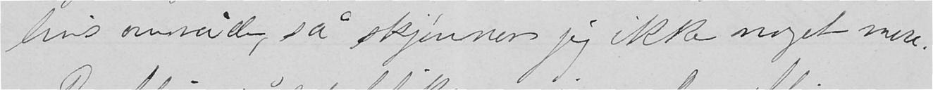livs område, så skjønner jeg ikke noget mere.
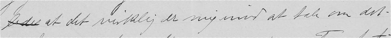ledes at det virkelig er mig imod at tale om det.
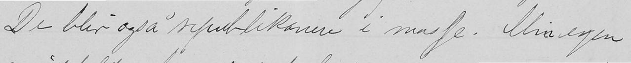De blir også republikanere i masse. Min egen
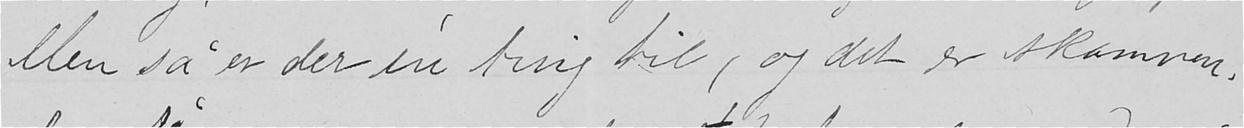Men så er der én ting til,og det er skammen,
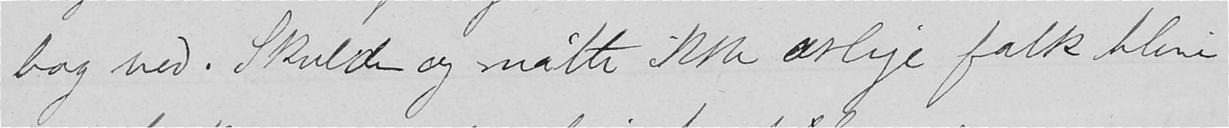bag ved. Skulde og måtte ikke ærlige folk blive
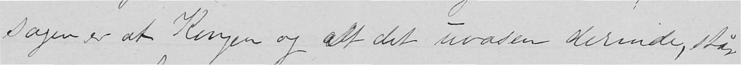sagen er at Kongen og alt det uvæsen derinde, står
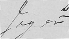Jeg er
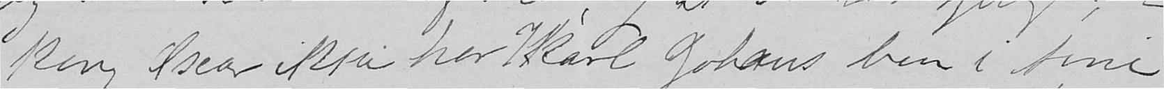kong Oscar ikke har Karl Johans ben i sine
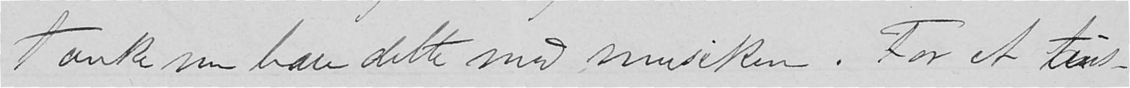Tænk nu bare dette med musiken. For et tus-
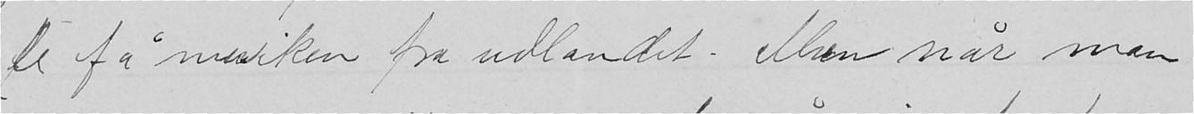le få musiken fra udlandet. Men når man
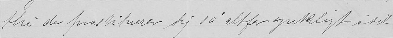thi de prostituerer sig så altfor opriktigt i sit
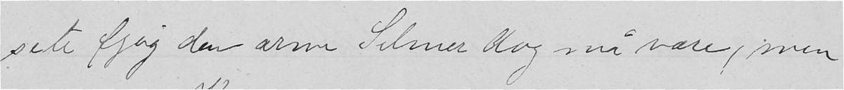sete fjog den arme Selmer dog må være, men
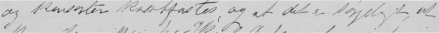og konsortér korstfæstes, og at det er sørgeligt, at
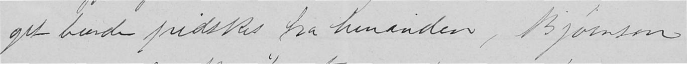get burde pidskes fra hinanden, Bjørnson
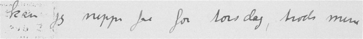kan jeg neppe faa før torsdag, trods mine
